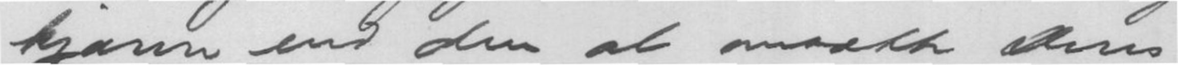kjærere end den at omsætte Deres
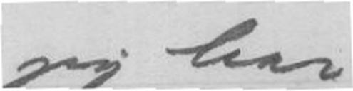jeg har
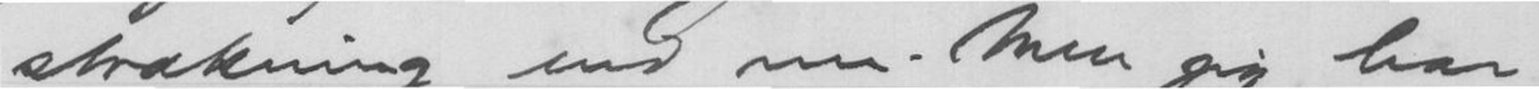strækning end nu. Men jeg har
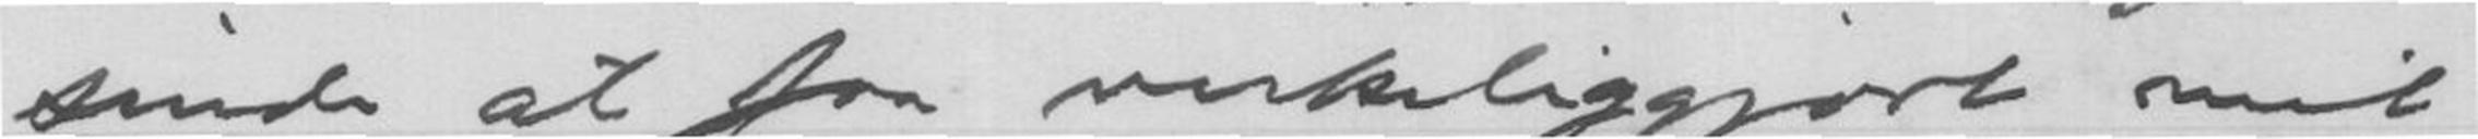sinde at faa virkeliggjort mit
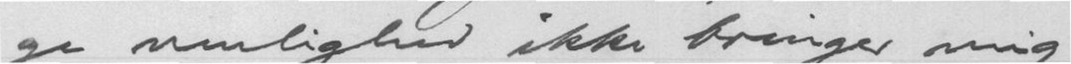ge mulighed ikke bringer mig
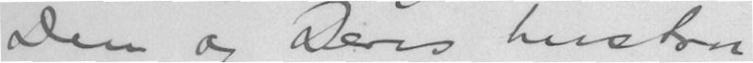Dem og Deres hustru
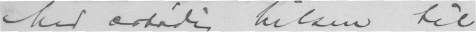Med ærbødig hilsen til
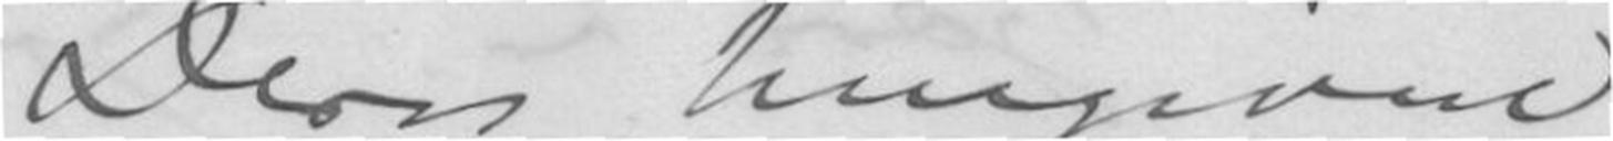Deres hengivne
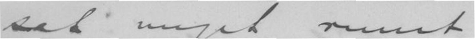sat meget rumt.
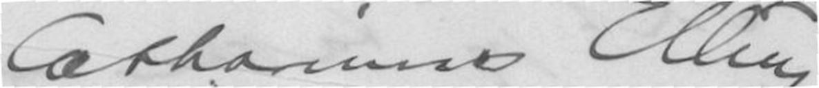Catharinius Elling
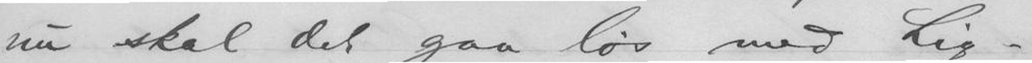nu skal det gaa løs med Lig-
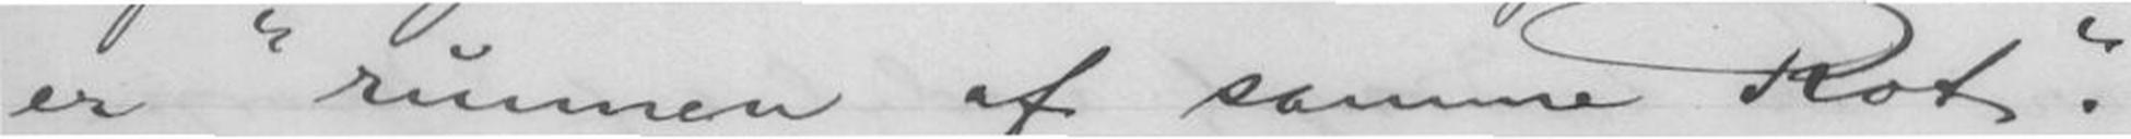er "runnen af samme Post".
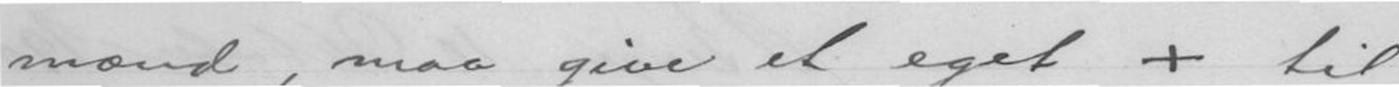mænd, maa give et eget + til
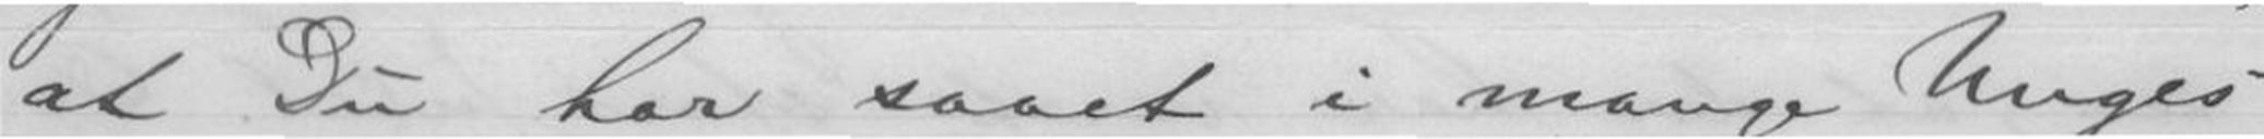at Du har saaet i mange Unges
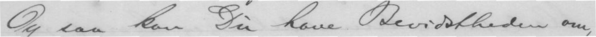Og saa kan Du have Bevidstheden om,
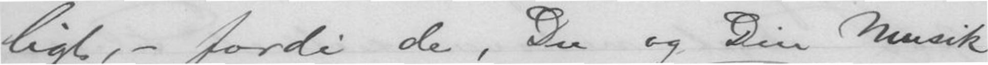ligt, - fordi de, Du og Din Musik
